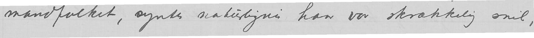mandfolket, syntes naturligvis han var skrækkelig snil,
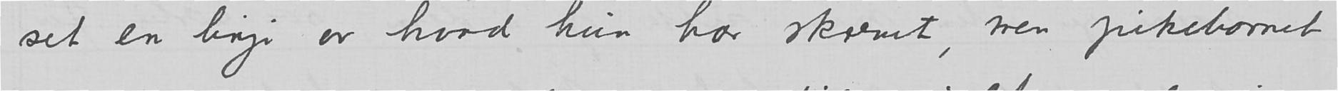set en linje av hvad hun har skrevet, men, pikebarnet
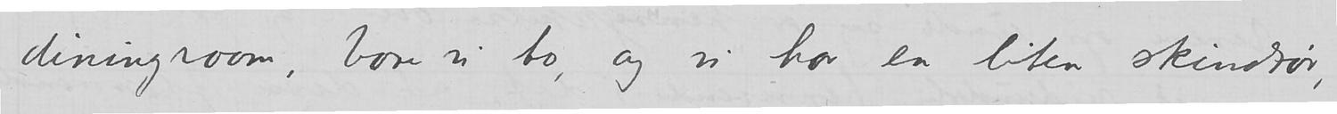diningroom, bare vi to, og vi har en liten skindtør,
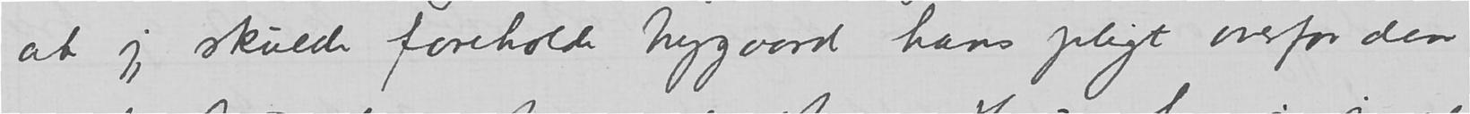at jeg skulde foreholde Nygaard hans pligt overfor den
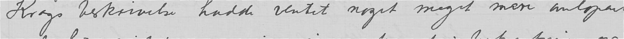Krags beskrivelser hadde ventet noget meget mere anløpent
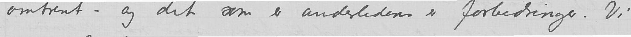omtrent – og det som er anderledens er forbedringer. Vi
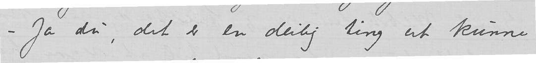– Ja du, det er en deilig ting at kunne
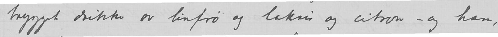brygget drikke av linfrø og lakris og citron – og han,
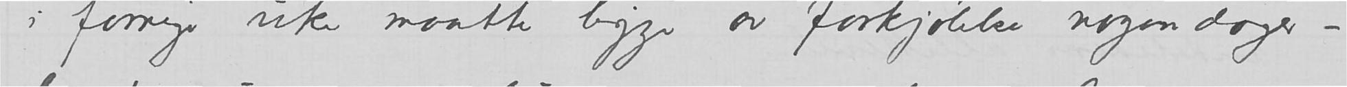i forrige uke maatte ligge av forkjølelse nogen dager –
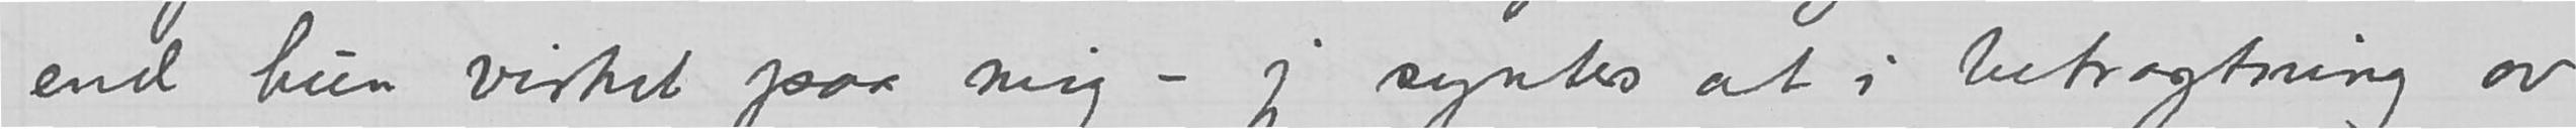end hun virket paa mig – jeg syntes at i betragtning av
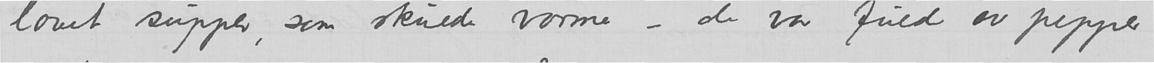lavet suppe, som skulde varme – den var fulde av pepper
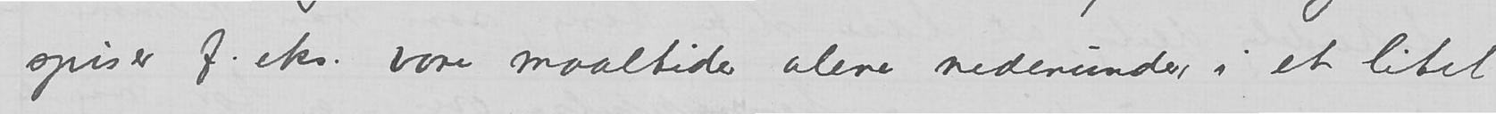spiser f. eks. vore maaltider alene nedenunder i et litet
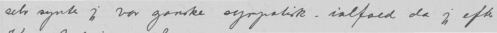selv syntes jeg var ganske sympatisk – ialfald da jeg efter
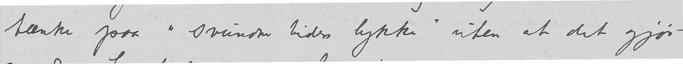tænke paa «svundne tiders» lykke uten at det gjør
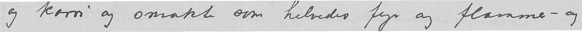og karri og smakte som helvedes fyr og flamme – og
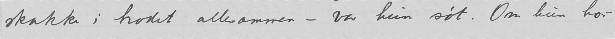skakke i hodet allesammen – var hun søt. Om hun har
